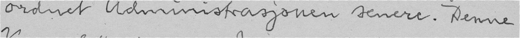ordnet Administrasjonen senere. Denne
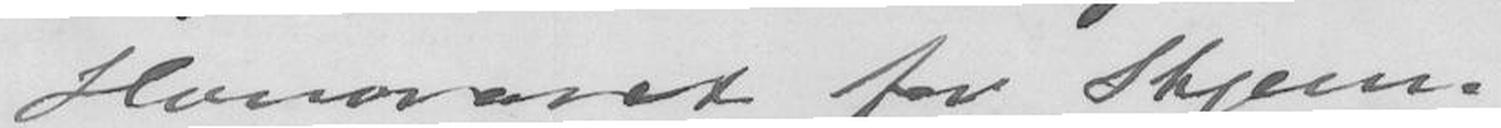Honoraret for Skjem-
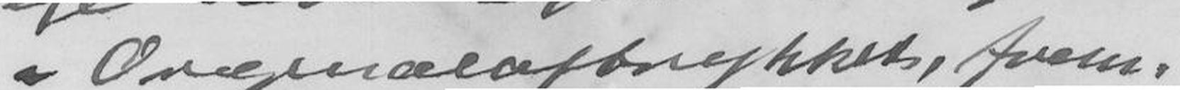i Oreginalaftrykket, frem-
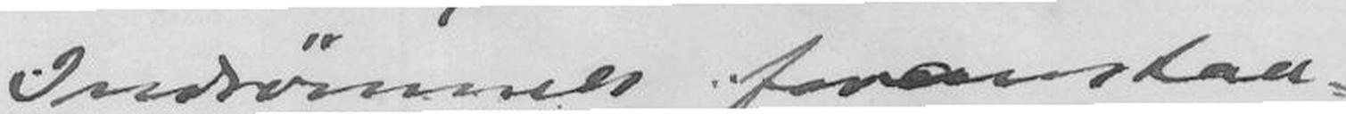Indrømmels foranstaa-
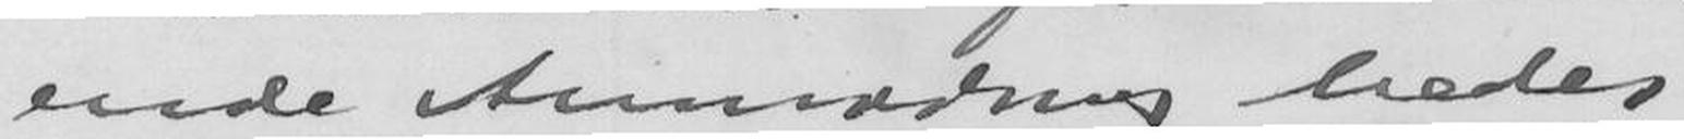ende Anmodning bedes
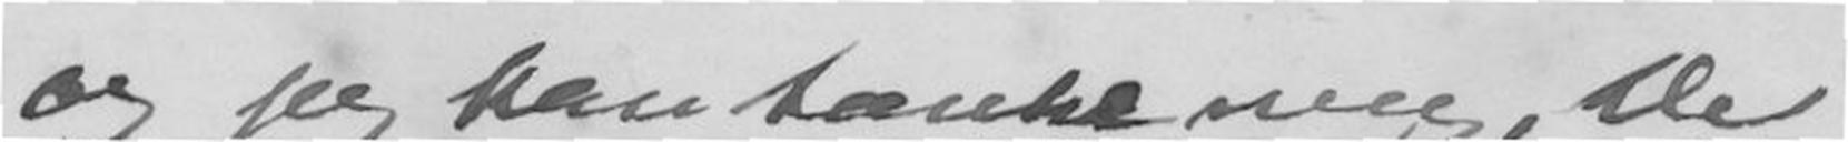og jeg kan tænke mig, De
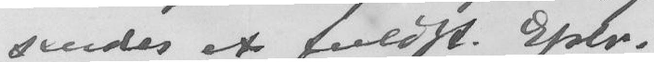sender et fuldst. Eplr.
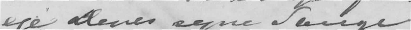eje Deres egne Sange
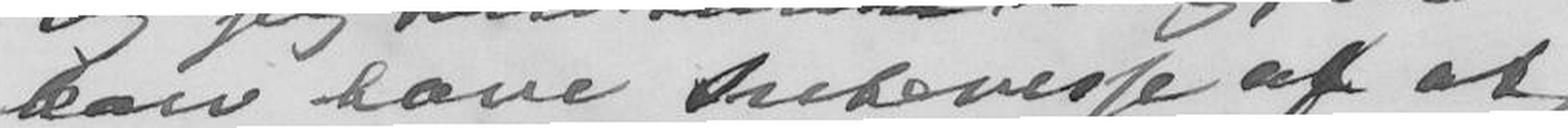kan have Interesse af at
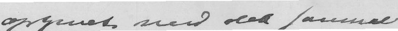opgivet med det samme
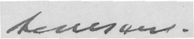tevisen.
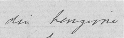din hengivne
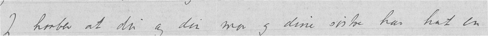Jeg haaber at du og din mor og dine søstre har hat en
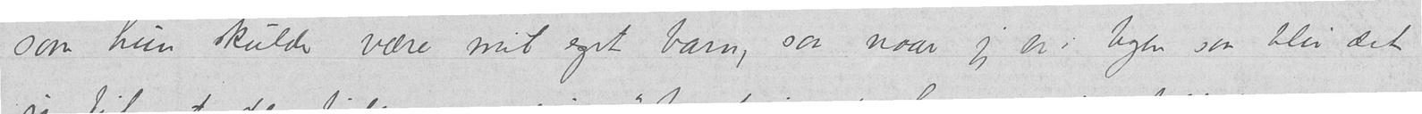som hun skulde være mit eget barn, saa naar jeg er i byen saa blir det
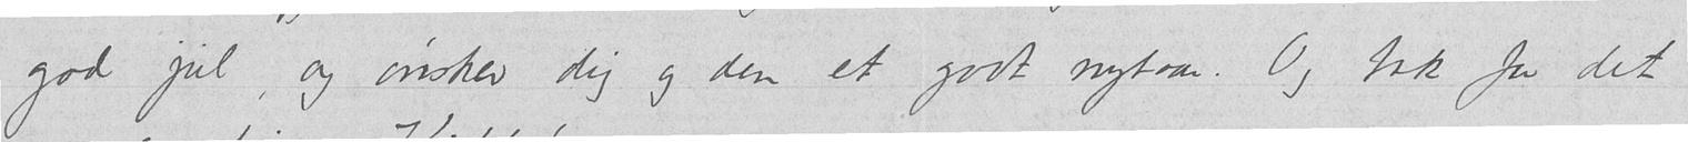god jul, og ønsker dig og dem et godt nytaar. Og tak for det
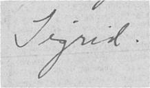Sigrid.
